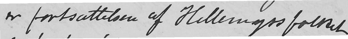er fortsættelsen af Hellemyrsfolket
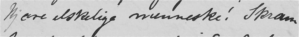kjære elskelige menneske! Skram
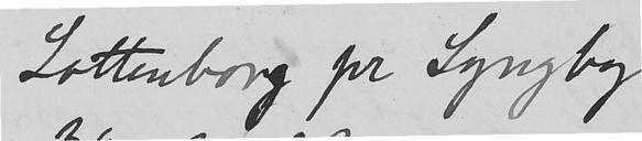Lottenborg pr Lyngby
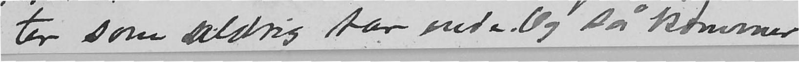ter som aldrig tar ende. Og så kommer
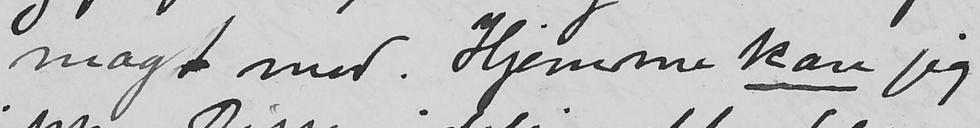magt med. Hjemme kan jeg
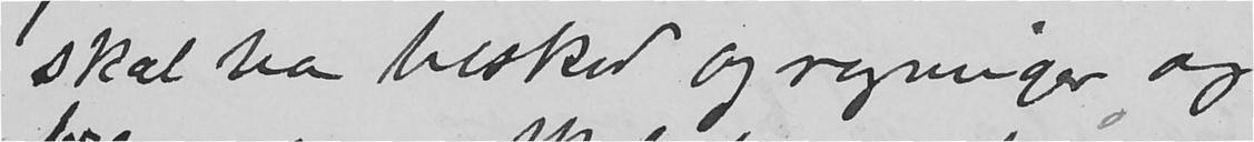skal ha besked og regninger og
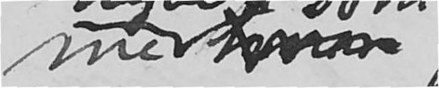me
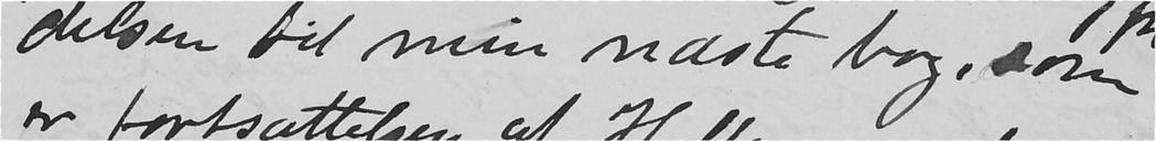delsen til min næste bog, som
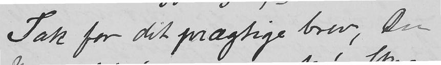Tak for dit prægtige brev, Du
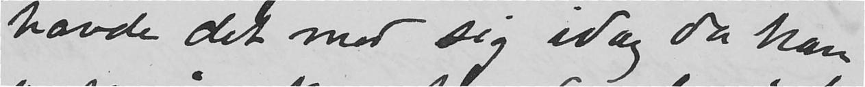havde det med sig idag da han
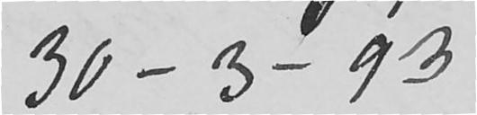30-3-93
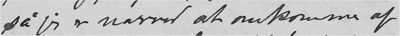så jeg er nærved at omkomme af
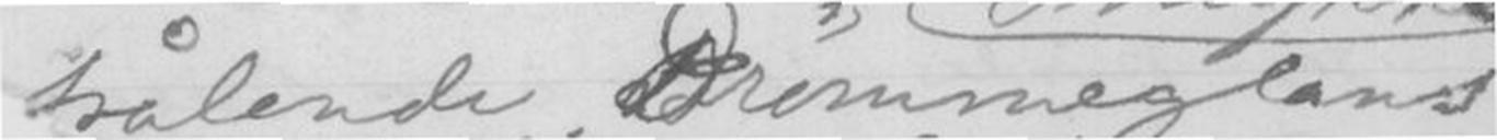trålende Drømmeglans
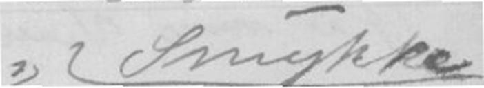Smykke
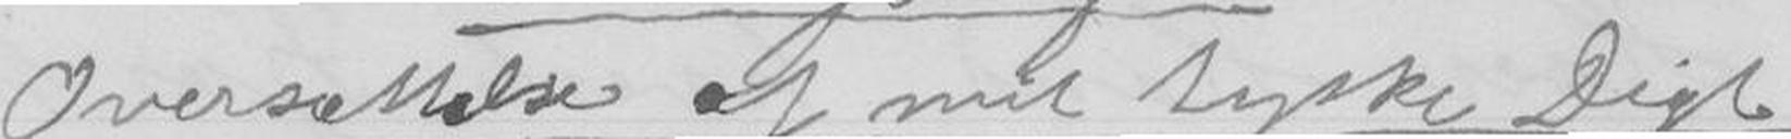Oversættelse af mit tyske Digt
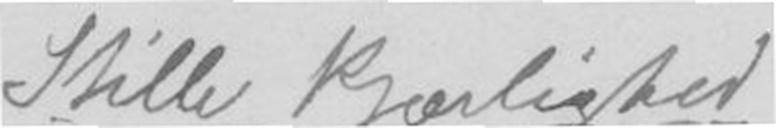Stille Kjærlighed
